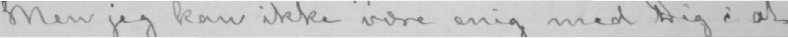Men jeg kan ikke være enig med Dig i at
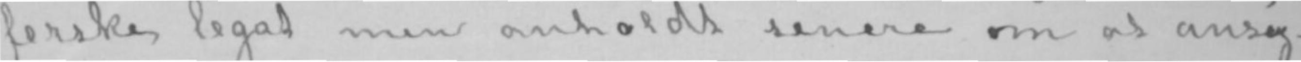ferske legat men anholdt senere om at ansøg-
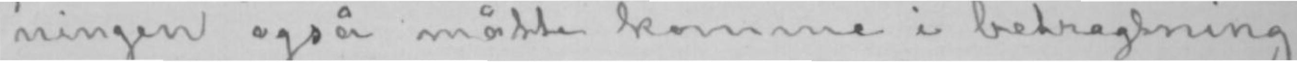ningen også måtte komme i betragtning
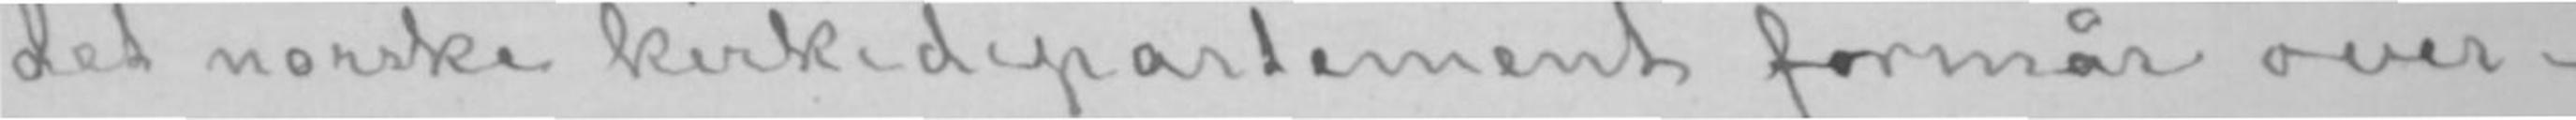det norske kirkedepartement formår over-
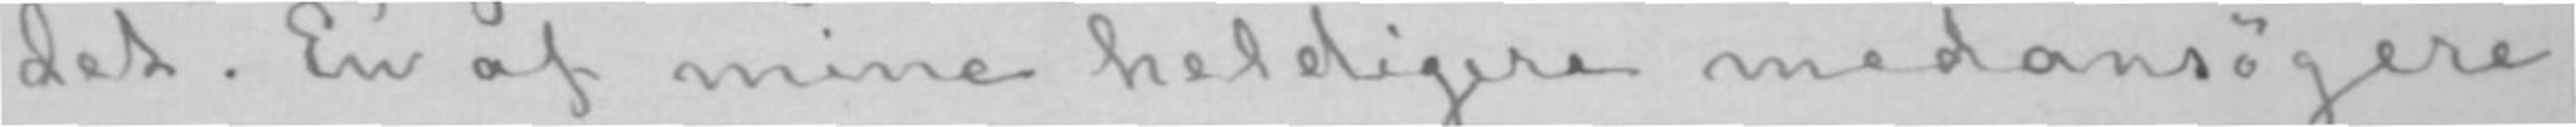det. En af mine heldigere medansøgere,
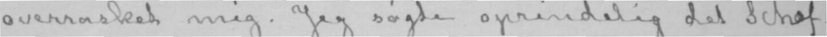overrasket mig. Jeg søgte oprindelig det Schæf-
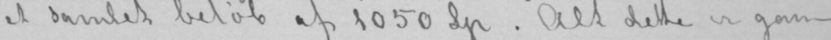et samlet beløb af 1050 Sp. Alt dette er gan-
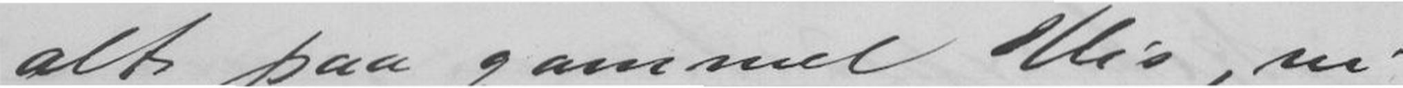alt paa gammel Vis, vi
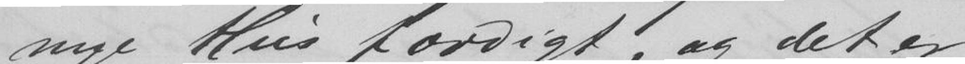nye Hus færdigt, og det er
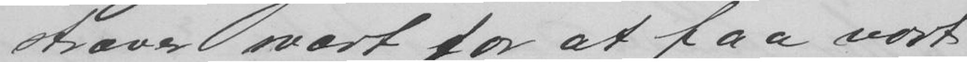stræver svært for at faa vort
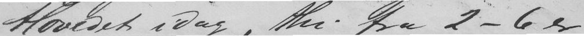Hovedet idag, Nei, fra 2-6 er
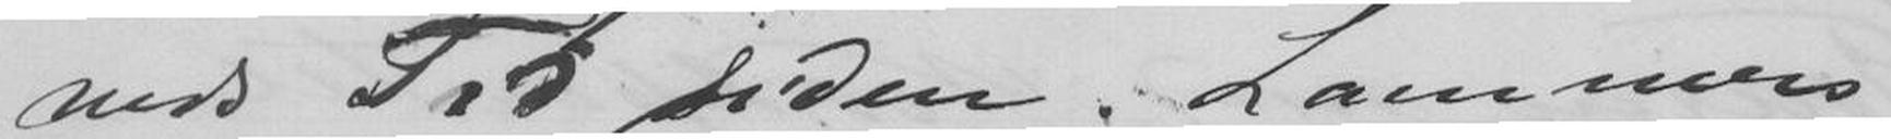neds Tid siden. Lammers
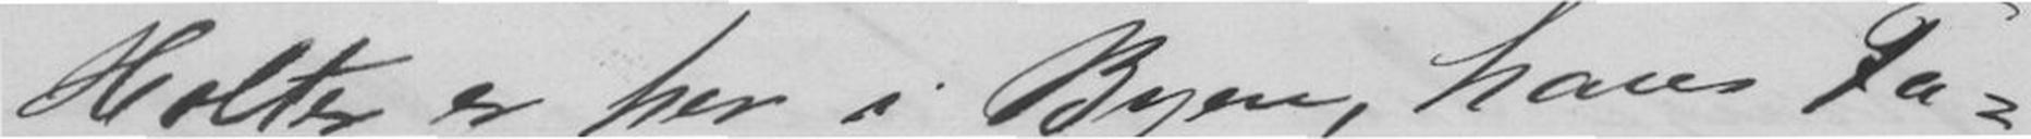Holter er her i Byen, hans Fa-
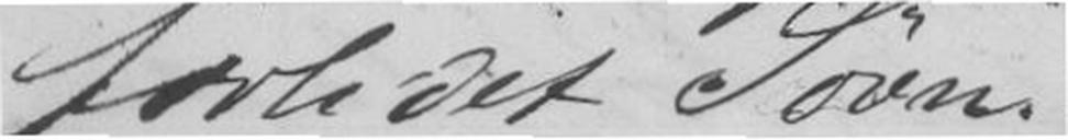forlidet Søvn.
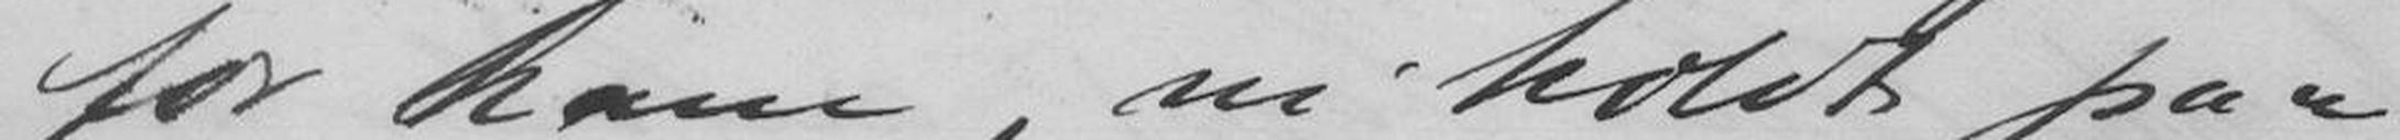for ham, vi holdt paa
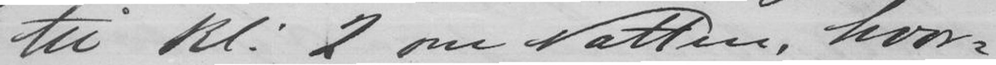til kl. 2 om Natten; hvor-
